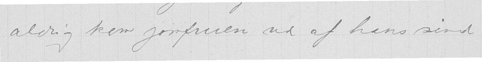aldrig kom jomfruen ud af hans sind
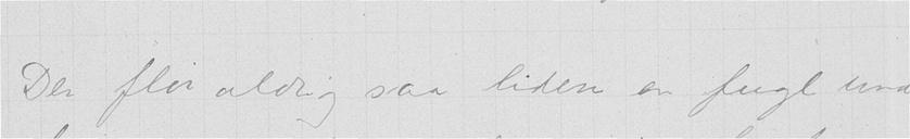Der fløj aldrig saa liden en fugl under ø
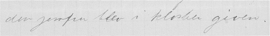den jomfru blev i kloster given.
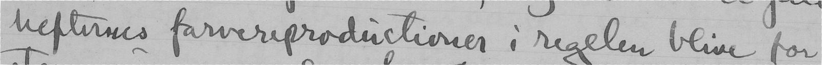hefternes farvereproductioner i regelen blive for
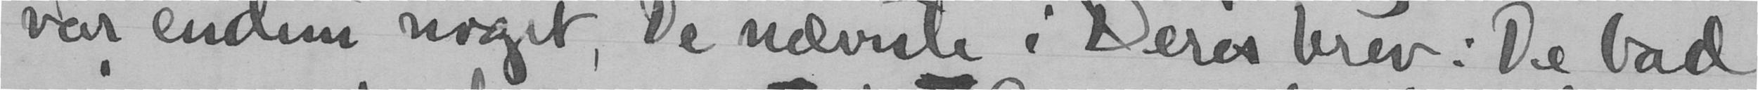var endnu noget, De nævnte i Deres brev: De bad
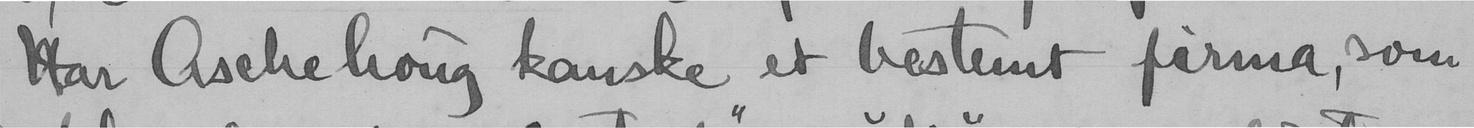Har Aschehaug kanske et bestemt firma, som
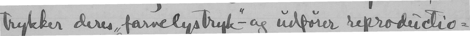trykker deres "farvelystryk" - og udfører reproductio-
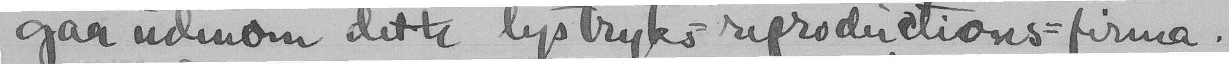gaa udmom dette lystryks-reproductions- firma.
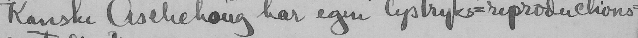Kanske Aschehaug har egen lystryks-reproductions-
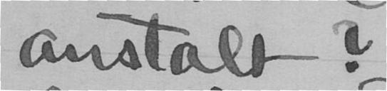anstalt?
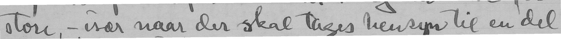store, - især naar der skal tages hensyn til en del
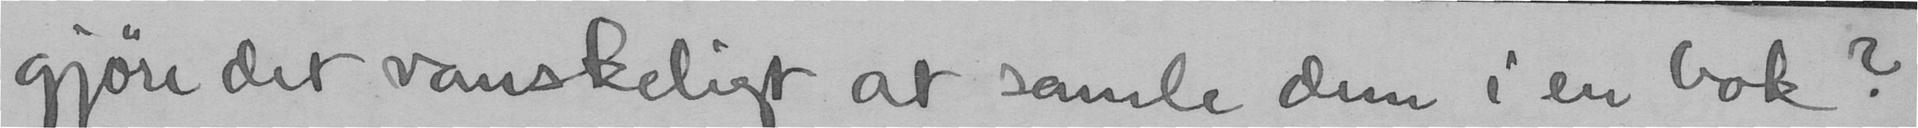gjøre det vanskeligt at samle dem i en bok?
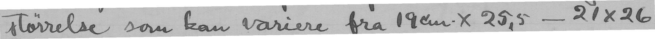størrelse som kan variere fra 19cm. x 25,5 - 21x26
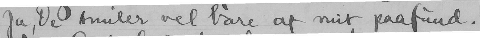Ja, De smiler vel bare af mit paafund.
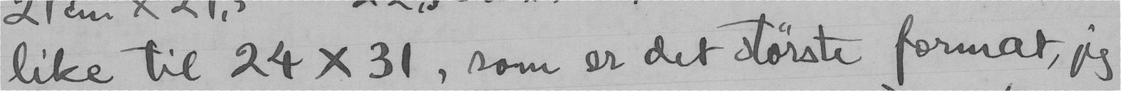like til 24x31, som er det største format, jeg
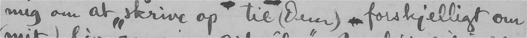mig om at "skrive op til (Dem)  forskjelligt om
